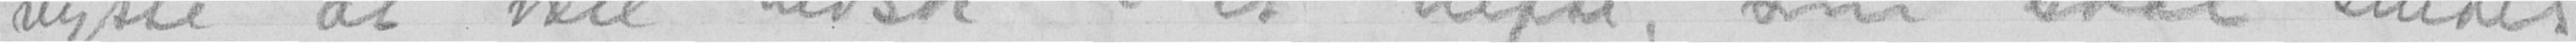nytte at være hadsk i et hefte, som skal sendes
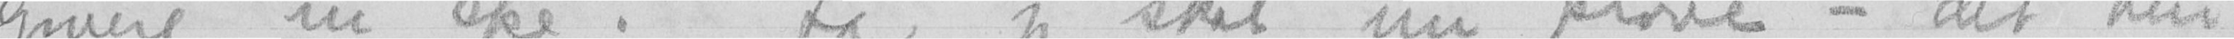givere in spe. Ja, jeg skal nu prøve - det blir
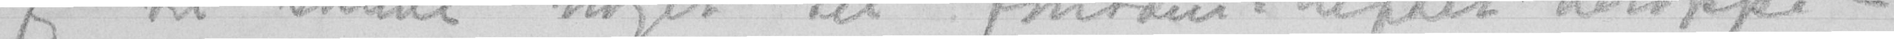Jeg vil skrive noget til fontæne-heftet heroppe-
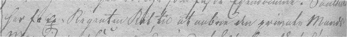har f: Ex: Regenten Ret til at aabne den private Mands
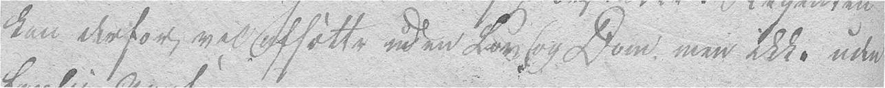kan derfor vel afsætte uden Lov og Dom, men ikke uden
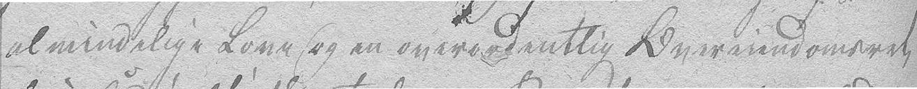almindelige Love og en overordentlig Overeiendomsret,
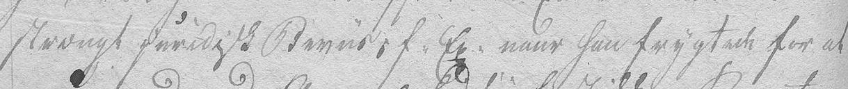strængt juridisk Beviis f: Ex: naar han frygtede for at
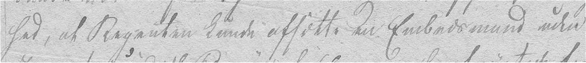hed, at Regenten kunde afsætte en Embedsmand uden
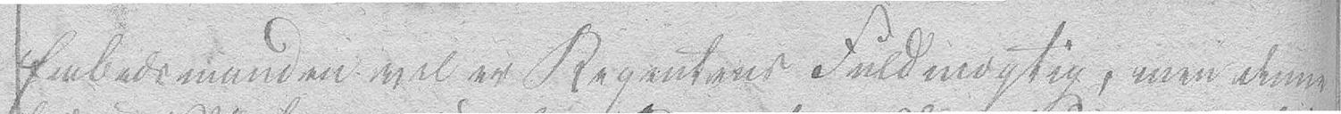Embedsmanden vel er Regentens Fuldmægtig, men denne
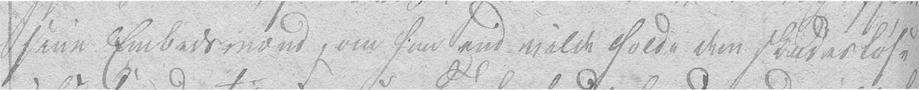sine Embedsmænd, om han end vilde holde dem skadesløse
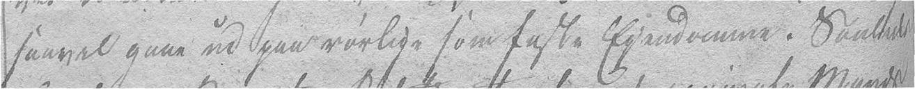saavel gaae ud paa rørlige som faste Eiendomme. Saaledes
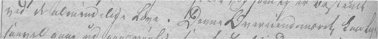ved de almindelige Love. Denne Overeiendomsret kan lige-
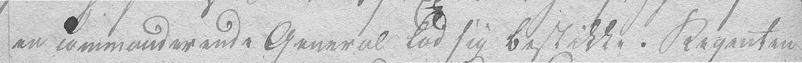en commanderende General lod sig bestikke. Regenten
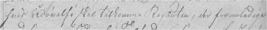hvis Udøvelse skal tilkomme Regenten, der foranlediges
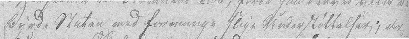byrde Staten med formange slige Understøttelser; der
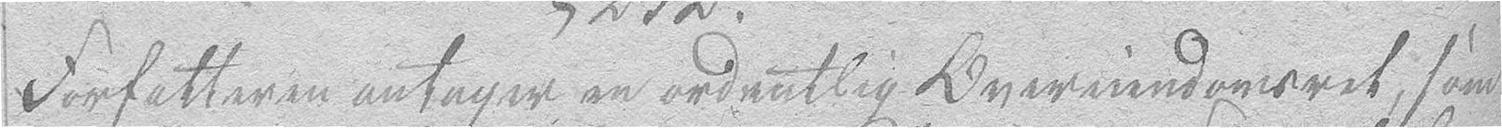Forfatteren antager en ordentlig Overeiendomsret, som
